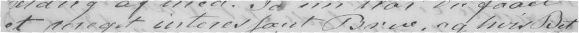et meget interessant Brev, og hvis Bet
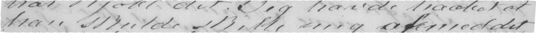han skulde skille mig af med det
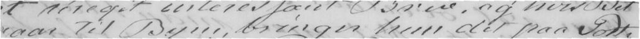aar til Byen, bringer hun det paa Post-
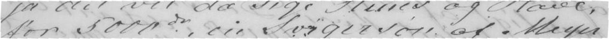for 5000do, en Svigerson af Meyer
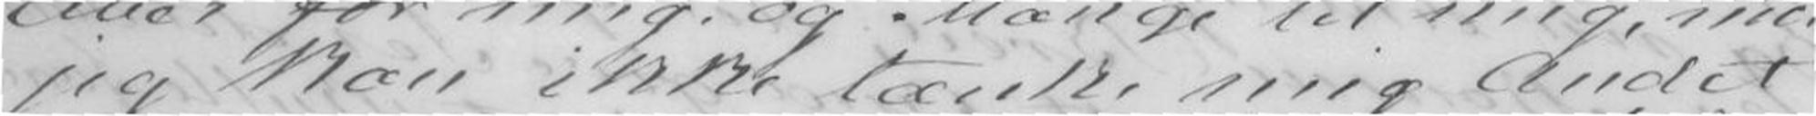jeg kan ikke tænke mig Andet
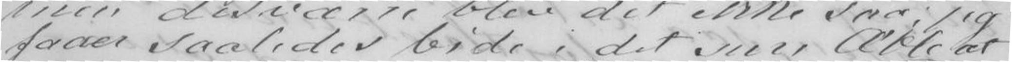fader saalider bide i det sure Æble at
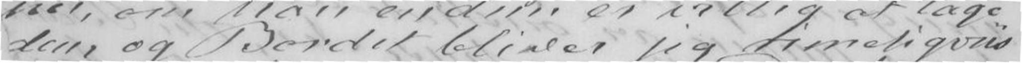dem og Bordet bliver jeg rimeligviis
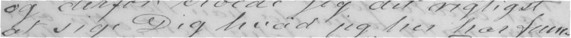at sige Dig hvad jeg her har frem-
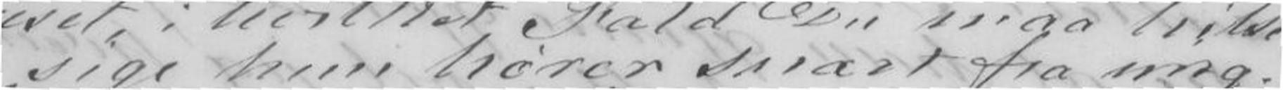sige hun hører snart fra mig.
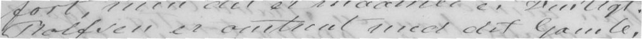Rolfsen er omtrent med det Gamle,
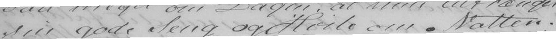sin gode Seng og Hvile om Natten.
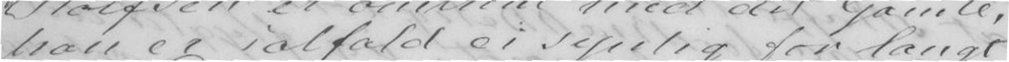han er ialfald ei synlig for langt
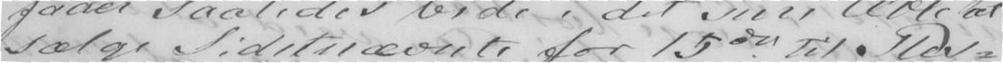sælge Sidstnævnte for 15do til Ples-
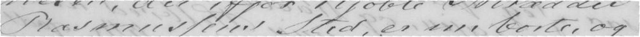Rasmussens Sted, er nu borte, og
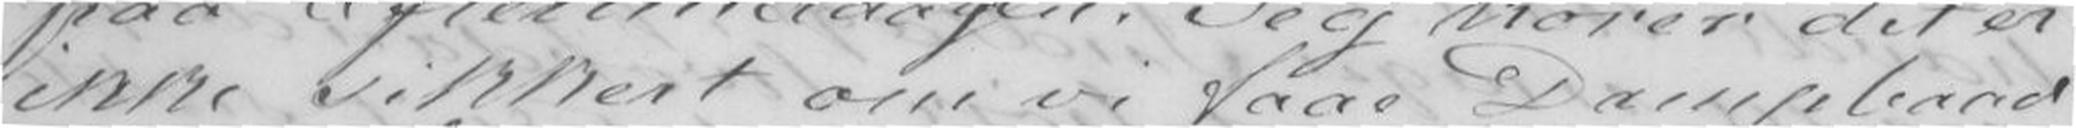ikke sikkert om vi faae Dampbaad
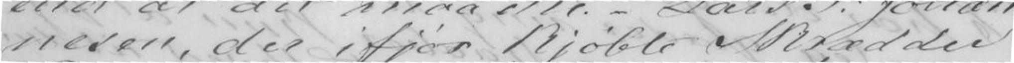nesen, der ifjor kjøbte Skrædder
